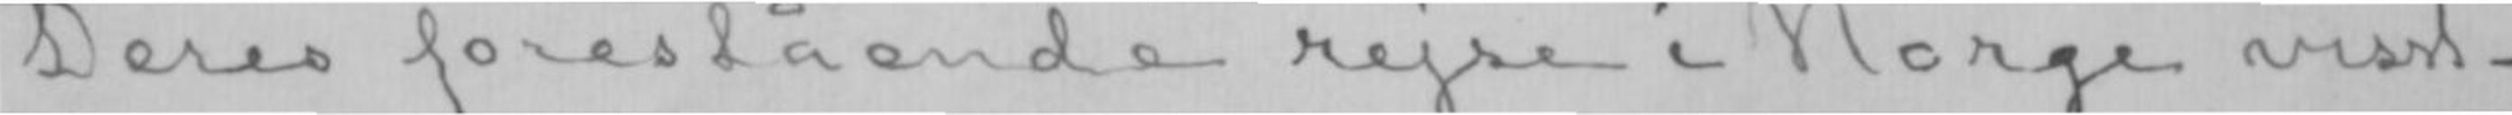Deres forestående rejse i Norge visst-
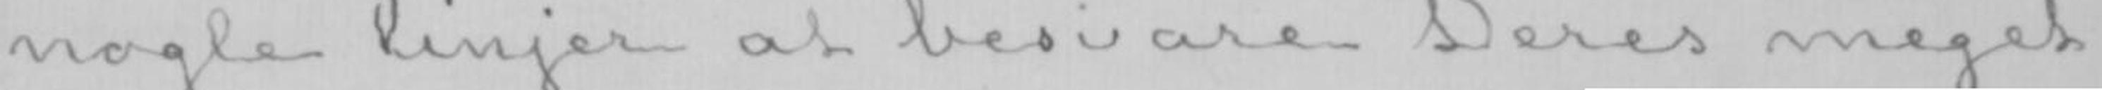nogle linjer at besvare Deres meget
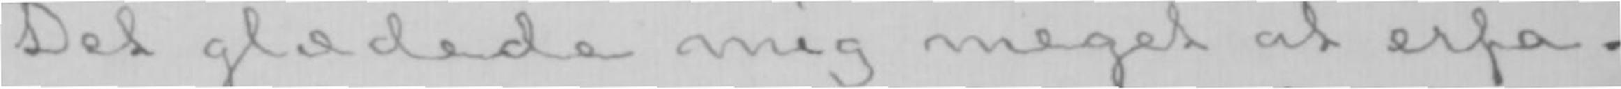Det glædede mig meget at erfa-
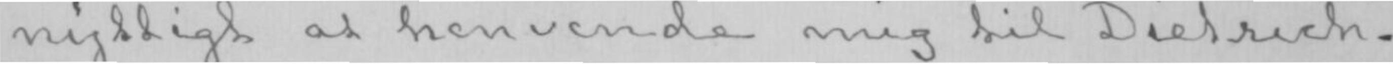nyttigt at henvende mig til Dietrich-
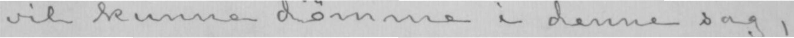vil kunne dømme i denne sag,
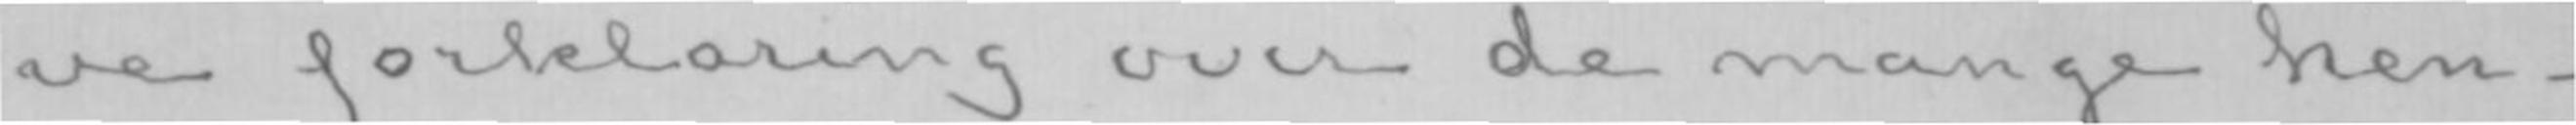ve forklaring over de mange hen-
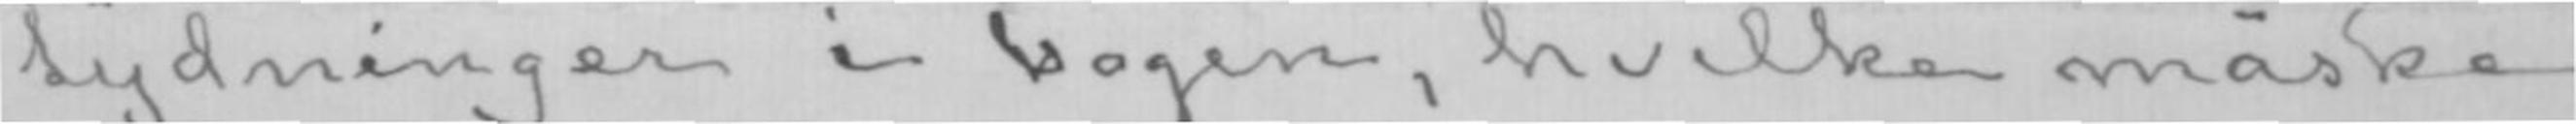tydninger i bogen, hvilke måske
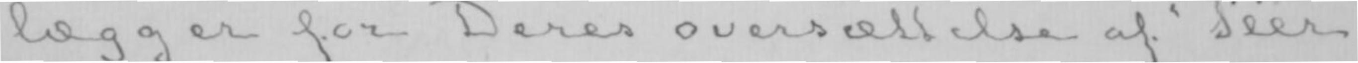lægger for Deres oversættelse af «Peer
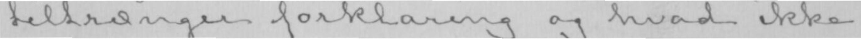tiltrænger forklaring og hvad ikke.
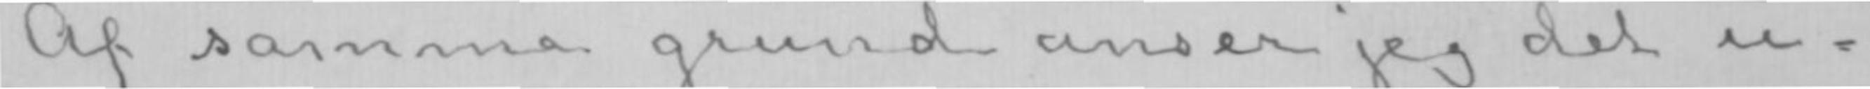Af samme grund anser jeg det u-
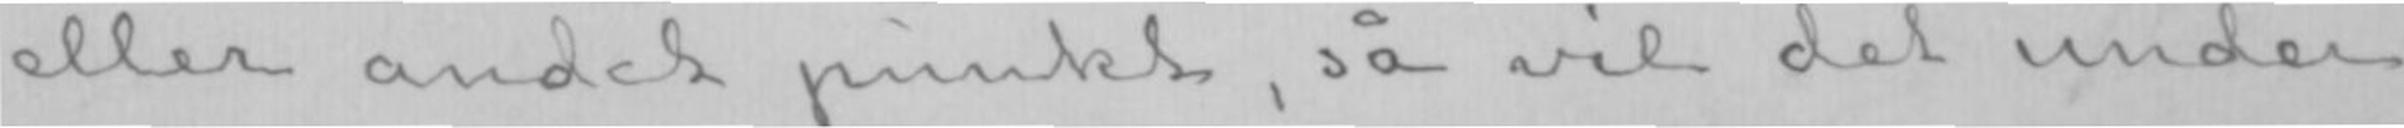eller andet punkt, så vil det under
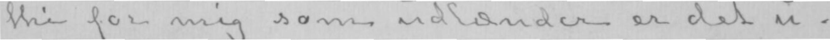thi for mig som udlænder er det u-
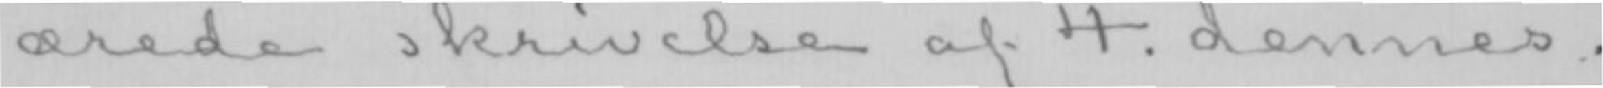ærede skrivelse af 4. dennes.
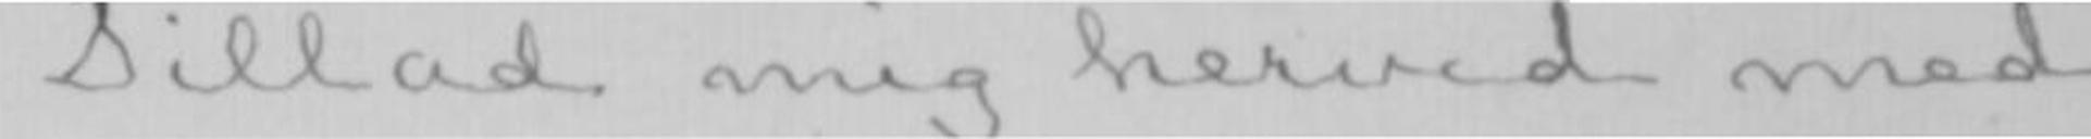Tillad mig herved med
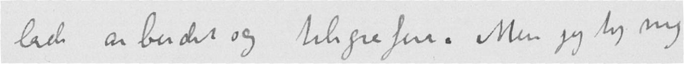lade arbeidet og telegrafere. Men jeg tog mig
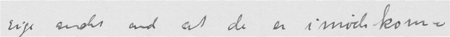sige andet end at de er imødekom-
